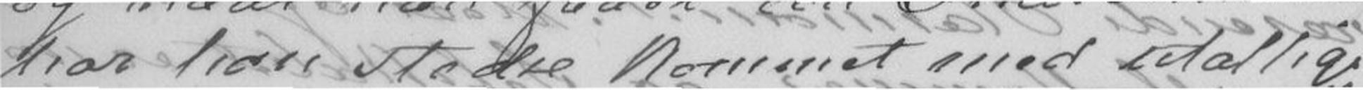har han stedse kommet med utallige
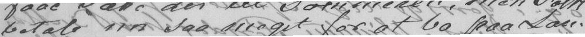betale nu saa meget for at bo paa Lan-
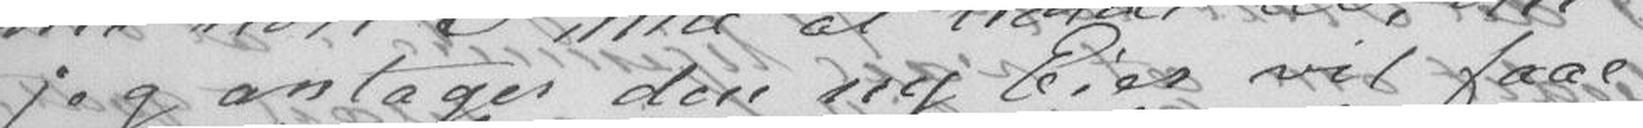jeg antager den ny Eier vil faae
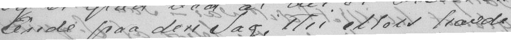Ende paa den Sag, thi ellers havde
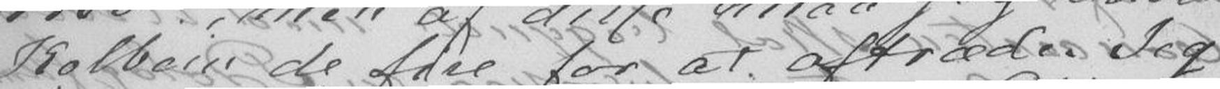Kolbein de fire for at aftræde. Jeg
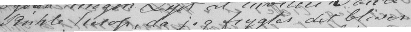Kühle herop, da jeg frygter det bliver
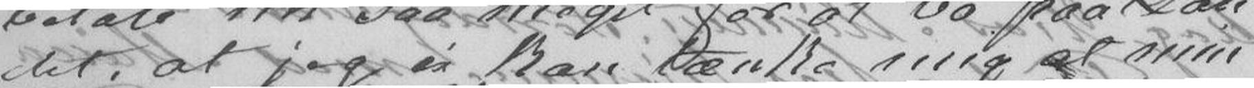det, at jeg ei kan tænke mig at min
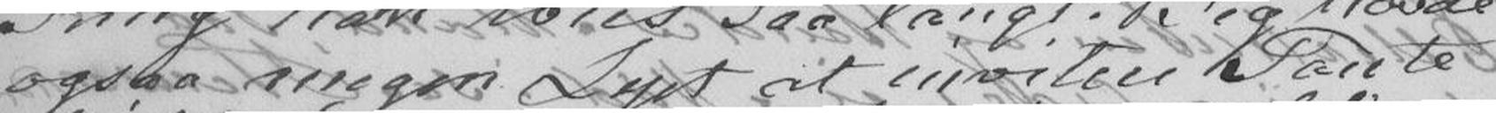ogsaa megen Lyst at invitere Tante
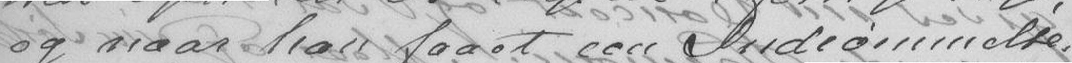og naar han faaet een Indrømmelse,
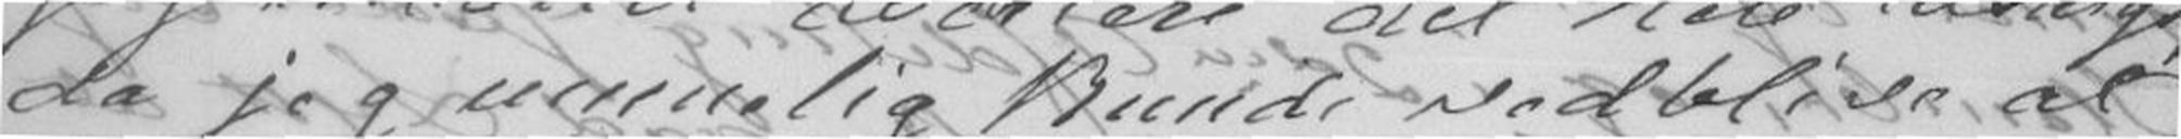da jeg umuelig kunde vedblive at
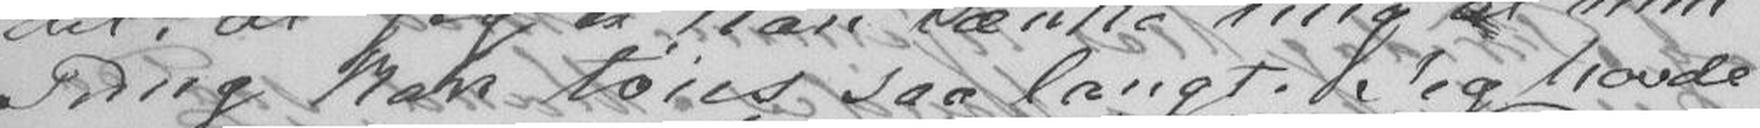Pung kan tøies saa langt. Jeg havde
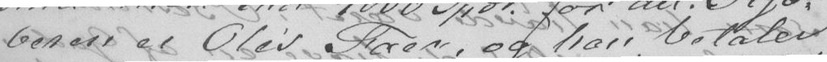beren er Ole's Faer, og han betaler
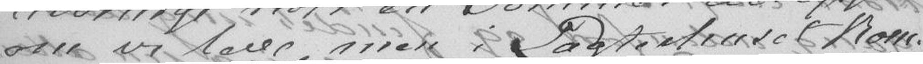om vi leve, men i Pagterhuset kom-
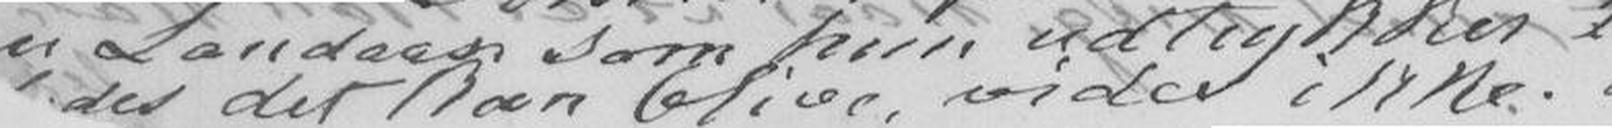des det kan blive vides ikke.
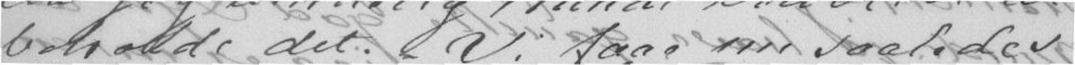beholde det. Vi faae nu saaledes
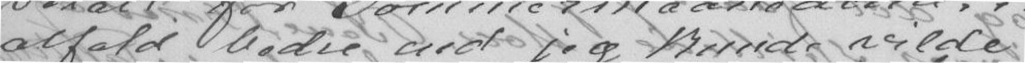alfalt bedre end jeg kunde vilde
